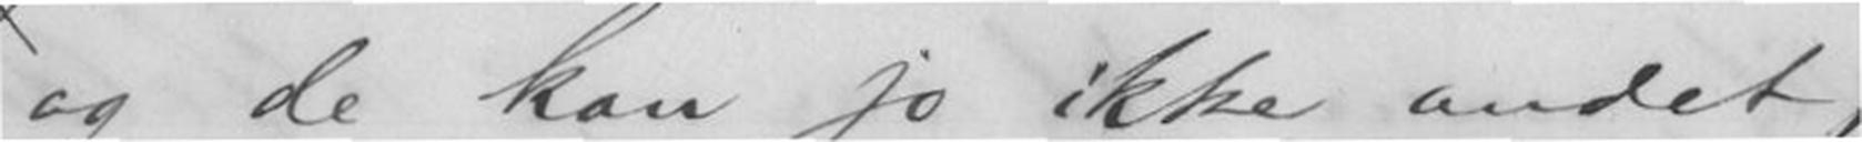og de kan jo ikke andet,
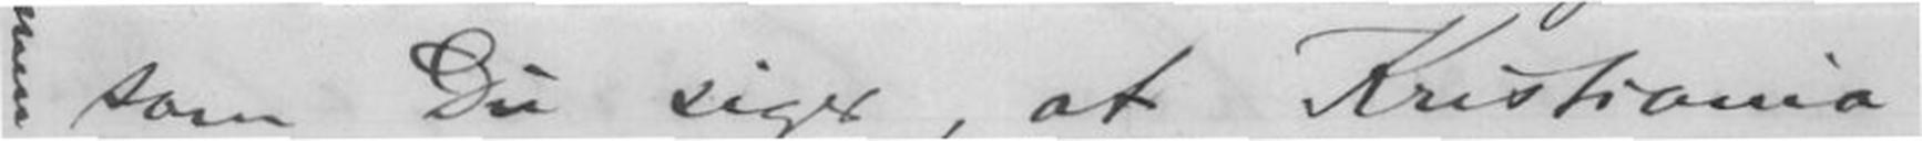som Du siger, at Kristiania-
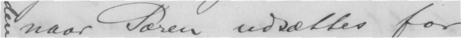naar Pæren udsættes for
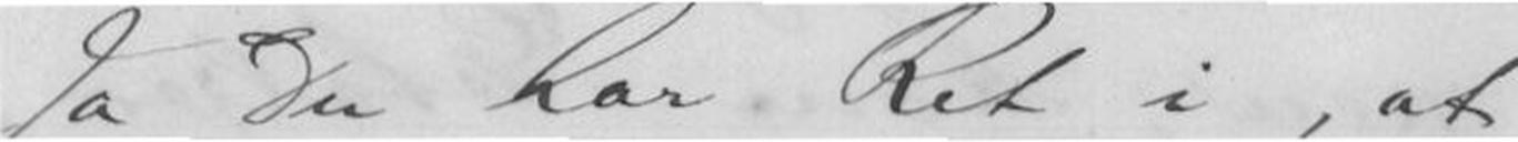Ja Du har Ret i, at
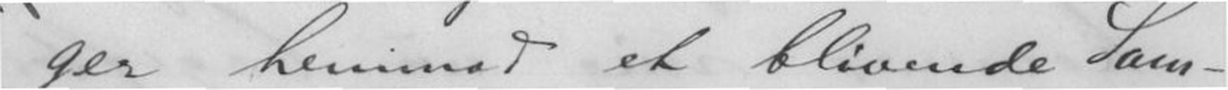ger henimod et blivende Sam-
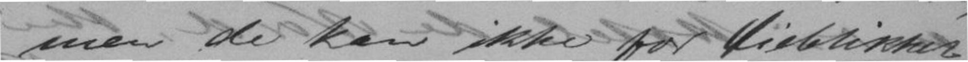men de kan ikke for Øieblikket
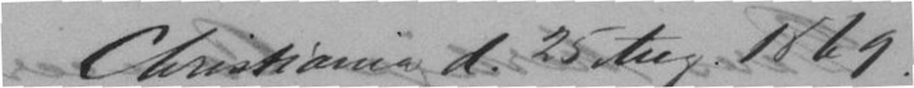Christiania d. 25 august 1869.
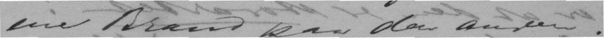ene Brand paa den anden;
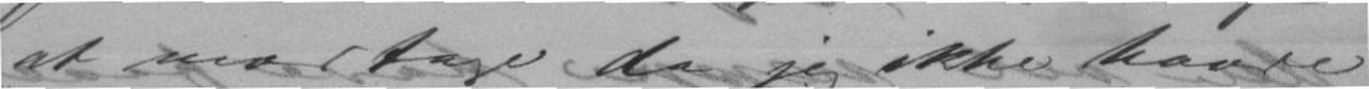at modtage da jeg ikke havde
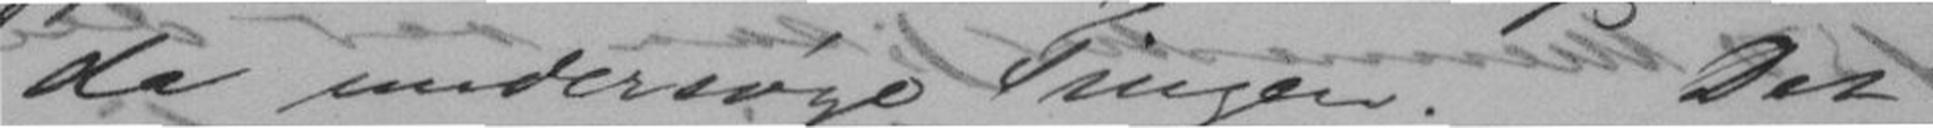da undersøge Tingen. Det
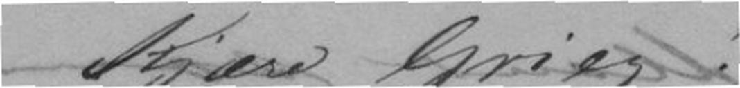Kjære Grieg!
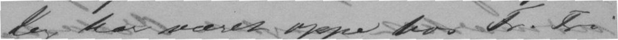Jeg har været oppe hos Fr. Fri-
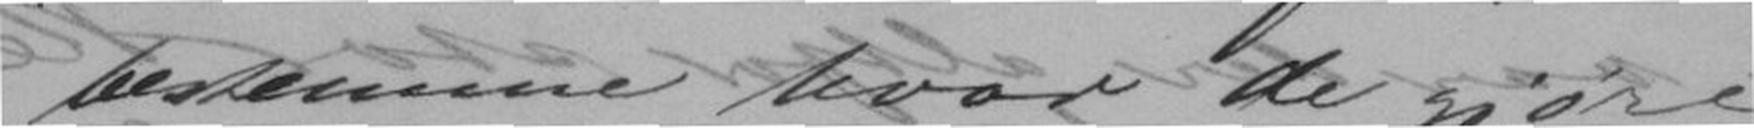bestemme hvor de gjøre
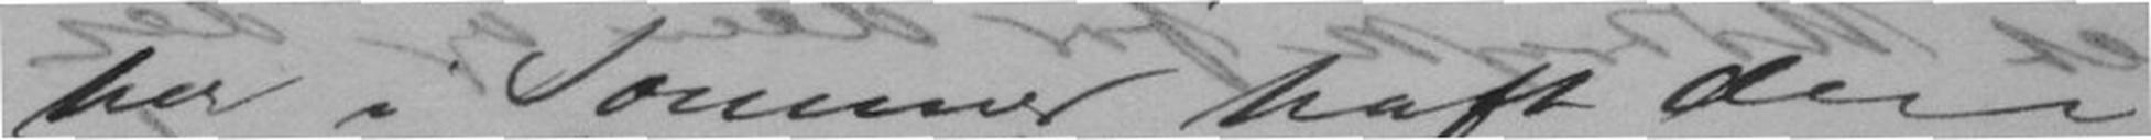her i Sommer haft den
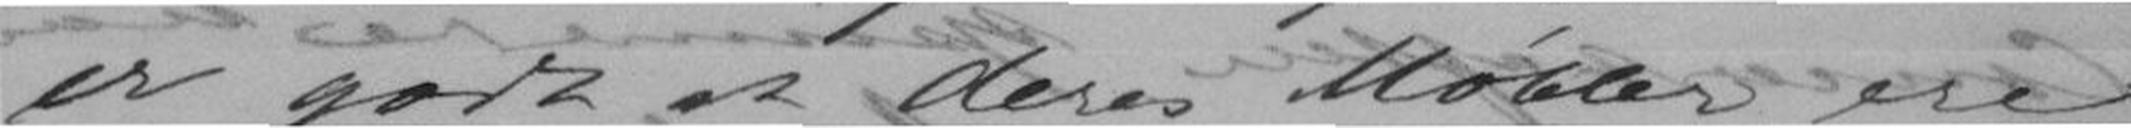er godt at deres Møbler ere
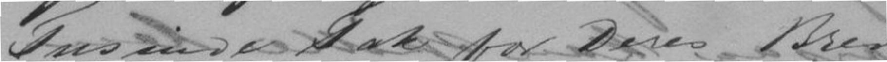Tusinde Tak for Deres Brev
